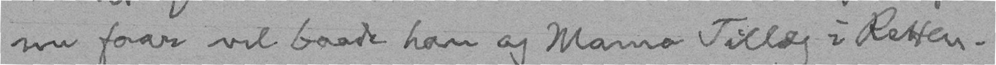nu faar vel baade han og Mama Tillæg i Retten.
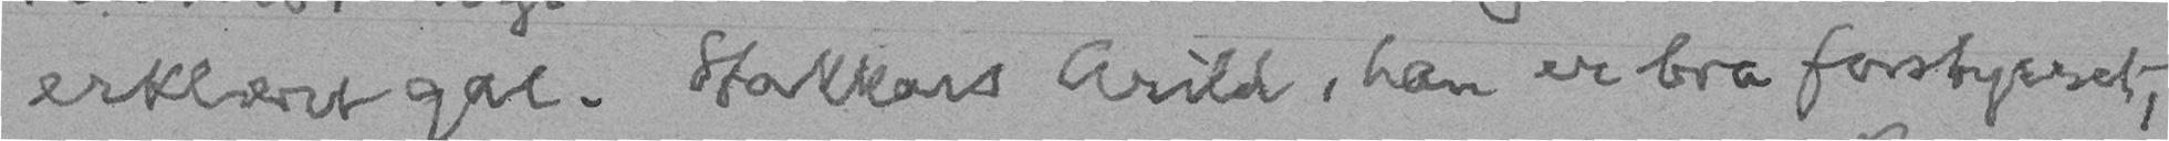erklæret gal. Stakkars Arild, han er bra forstyrret,
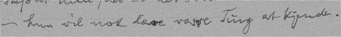- hun vil nok lære værre Ting at kjende.
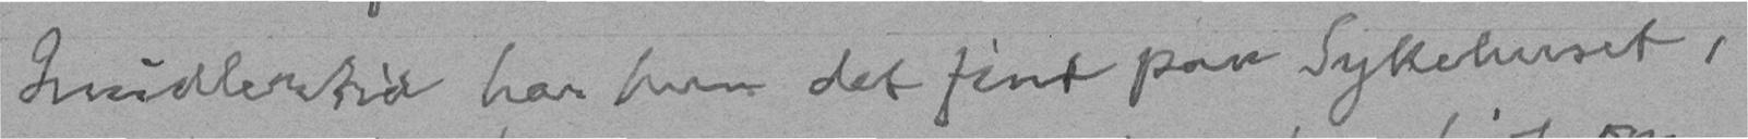Imidlertid har hun det fint paa Sykehuset,
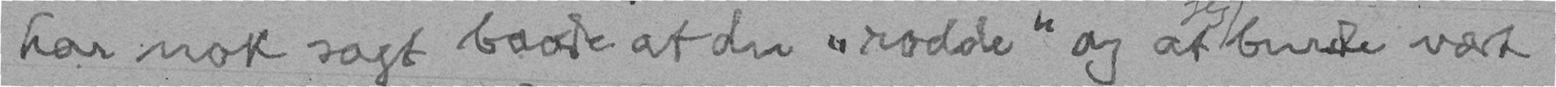har nok sagt baade at du "rodde" og at burde vært
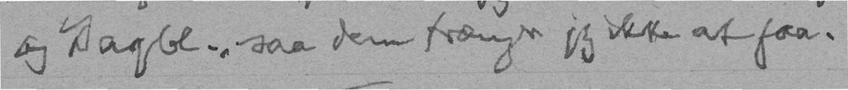og Dagbl., saa dem trænger jeg ikke at faa.
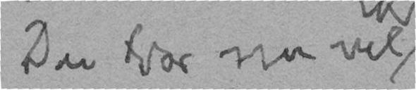Du tror nu vel
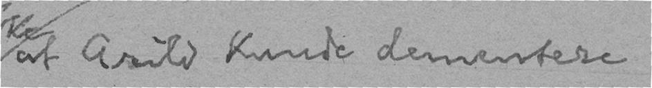at Arild kunde dementere
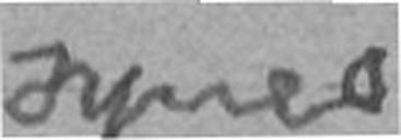synes
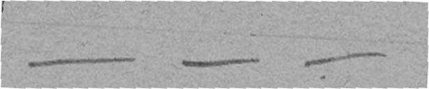- - -
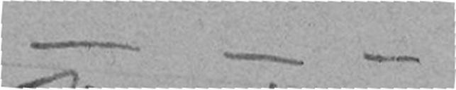- - -
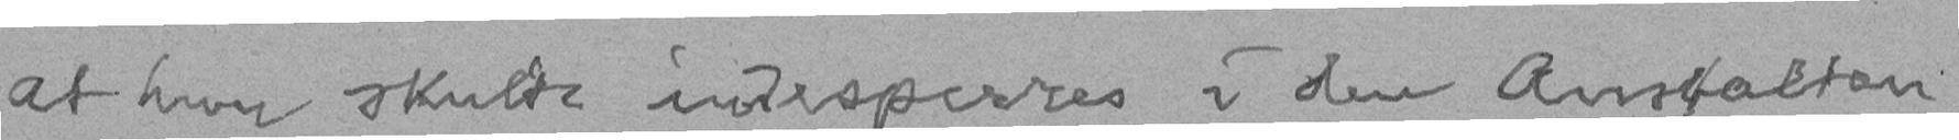at hun skulde indesperres i den Anstalten
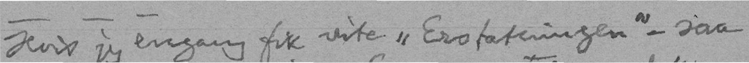Hvis jeg engang fik vite "Erstatningen" - saa
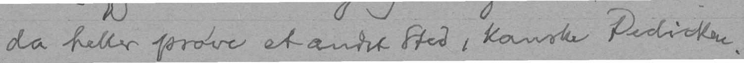da heller prøve et andet Sted, kanske Dedicken.
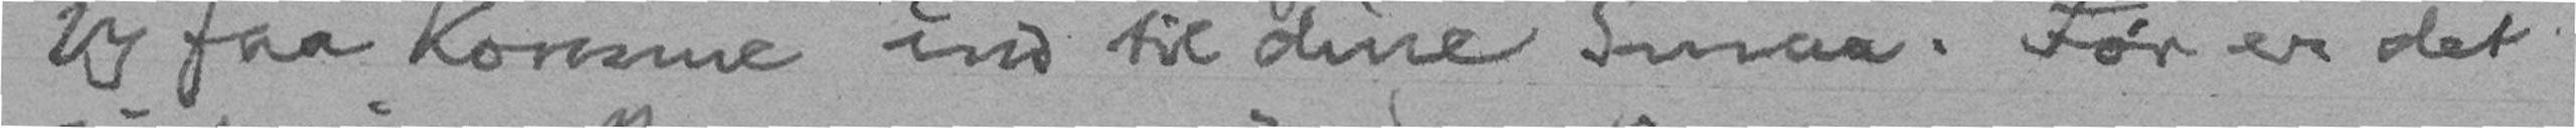jeg faa komme ind til dine Smaa. Før er det
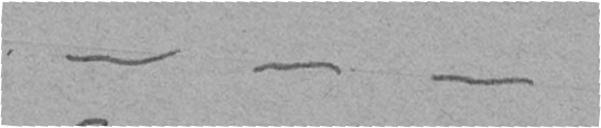- - -
